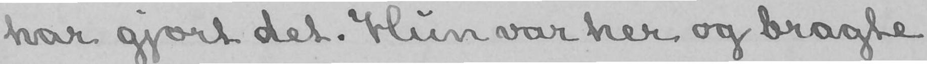har gjort det. Hun var her og bragte
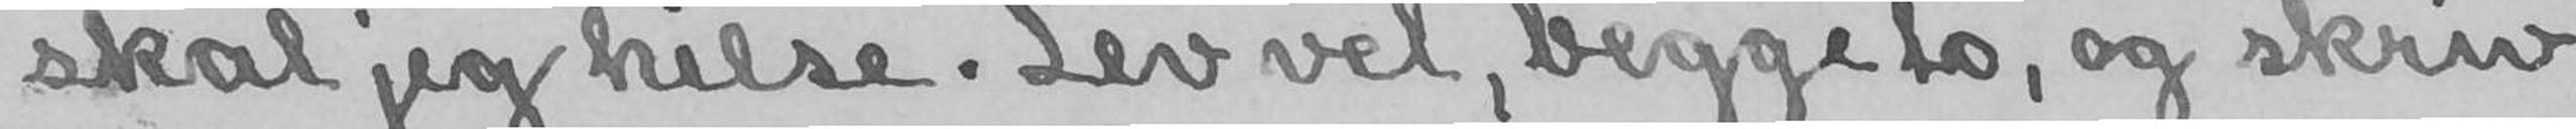skal jeg hilse. Lev vel, begge to, og skriv
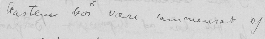kastene bør være sammensat af
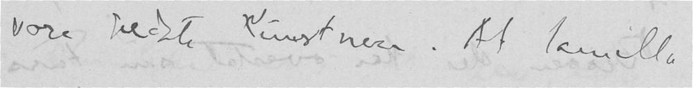vore bedste Kunstnere. At Camilla
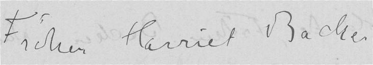Frøken Harriet Bacher
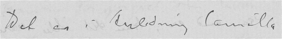Det er i Anledning Camilla
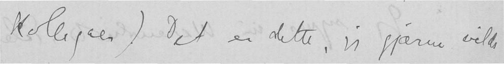Kollegaer) Det er dette, jeg gjerne vilde
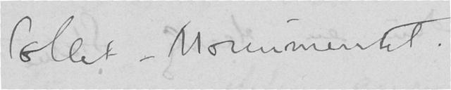Collet - Monumentet.
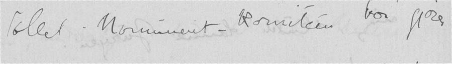Collet-Monument-Komiteen bør gjøre
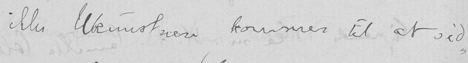ikke Ukunstnere kommer til at sid-
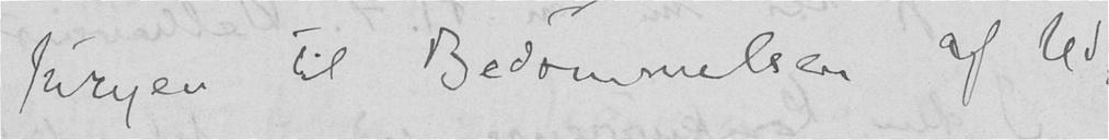Juryen til Bedømmelsen af Ud-
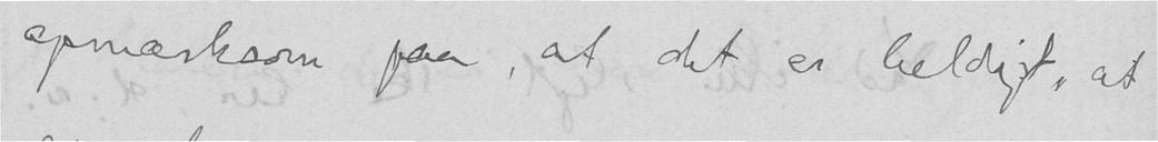opmærksom paa, at det er heldigt, at
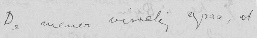De mener visselig ogsaa, at
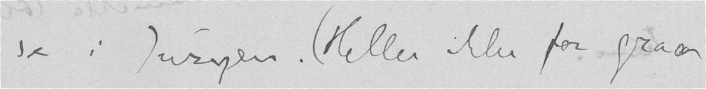de i Juryen. (Heller ikke for graa
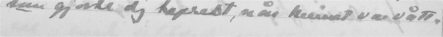som gjorde dig taprest, når kunnet var vått.
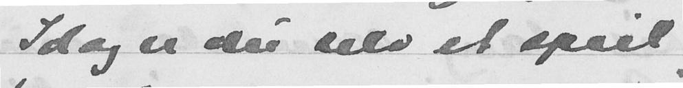Idag er der selv et speil
